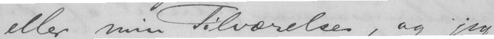eller min Tilværelse, og jeg
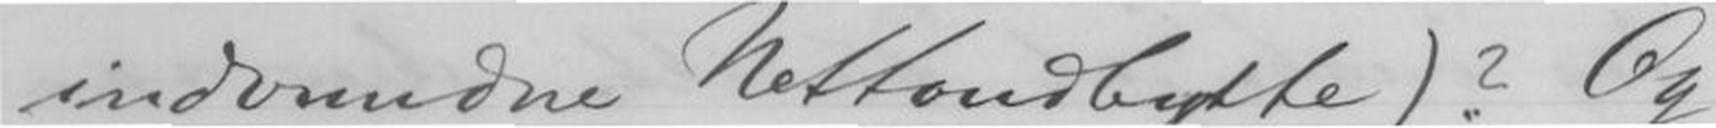indvundne Nettoudbytte)? Og
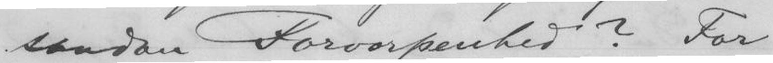saadan Forvorpenhed? For
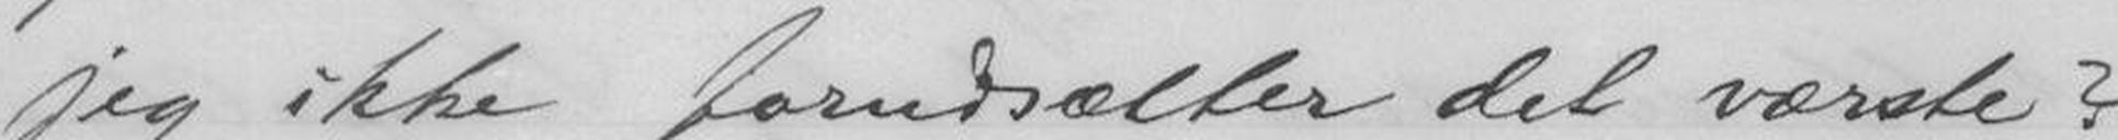jeg ikke forudsætter det værste?
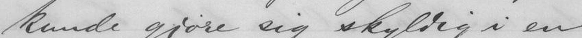kunde gjøre sig skyldig i en
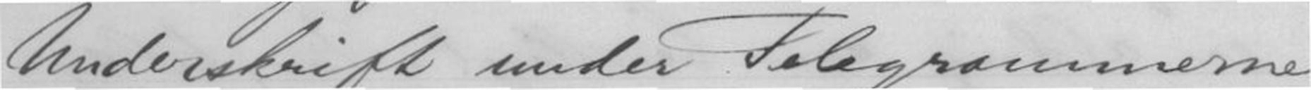Underskrift under Telegrammerne
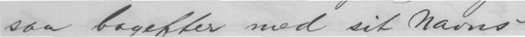saa bagefter med sit Navns
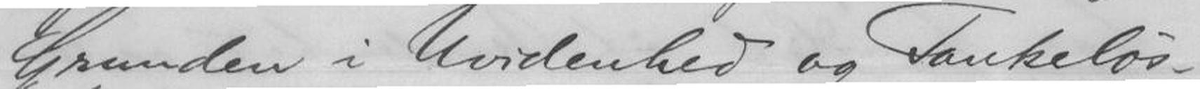Grunden i Uvidenhed og Tankeløs-
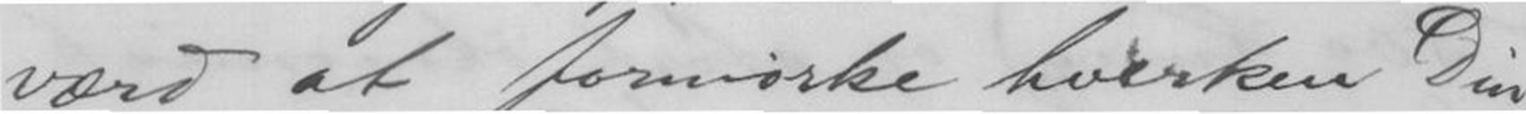værd at formørke hverken Din
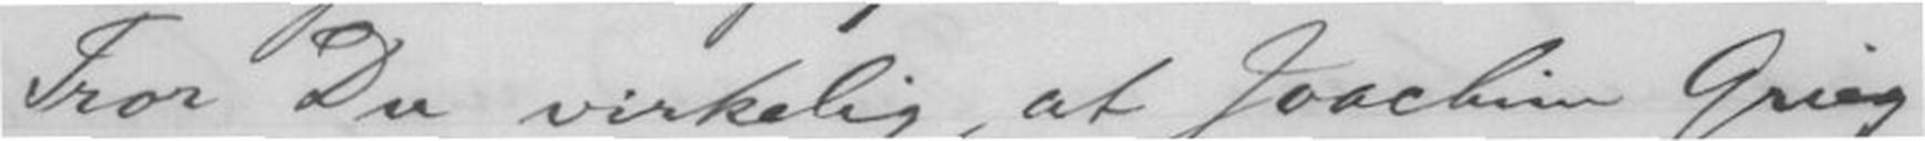Tror Du virkelig, at Joachim Grieg
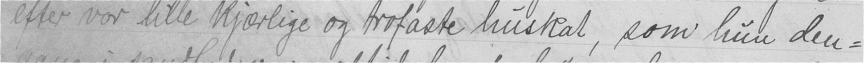efter vor lille kjærlige og trofaste huskat, som hun den-
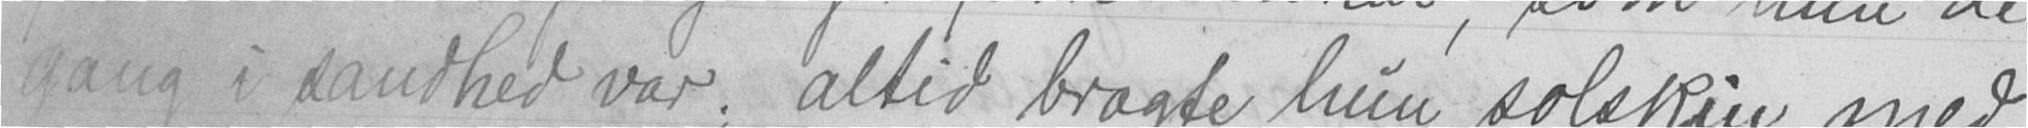gang i sandhed var; altid bragte hun solskin med
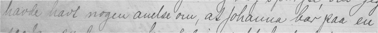havde havt nogen anelse om, at Johanna bar paa en
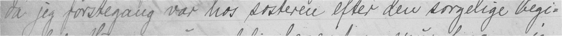da jeg førstegang var hos søsteren efter den sørgelige begi-
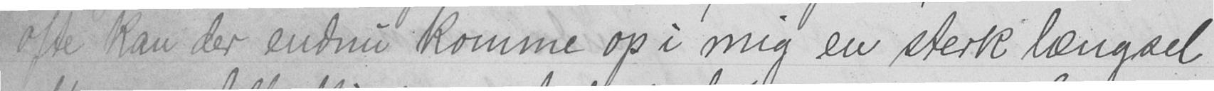ofte kan der endnu komme op i mig en sterk længsel
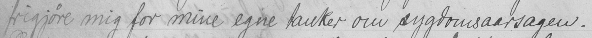frigjøre mig for mine egne tanker om sygdomsaarsagen.
